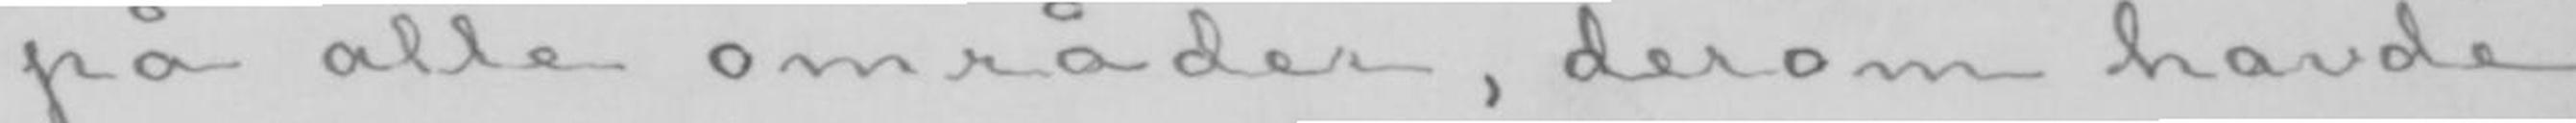på alle områder, derom havde
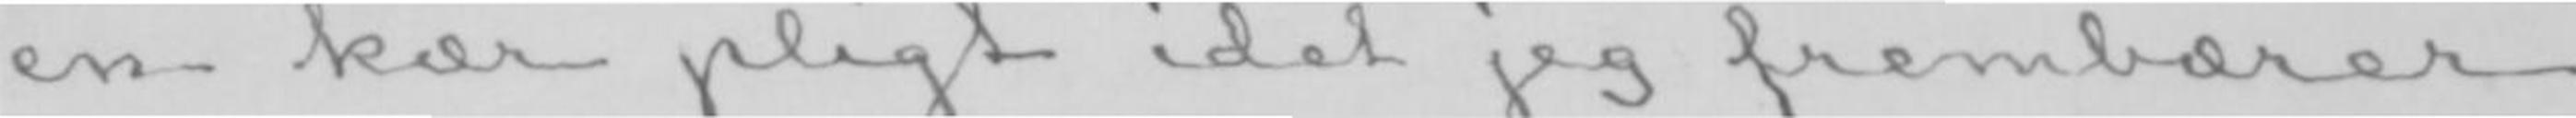en kær pligt idet jeg frembærer
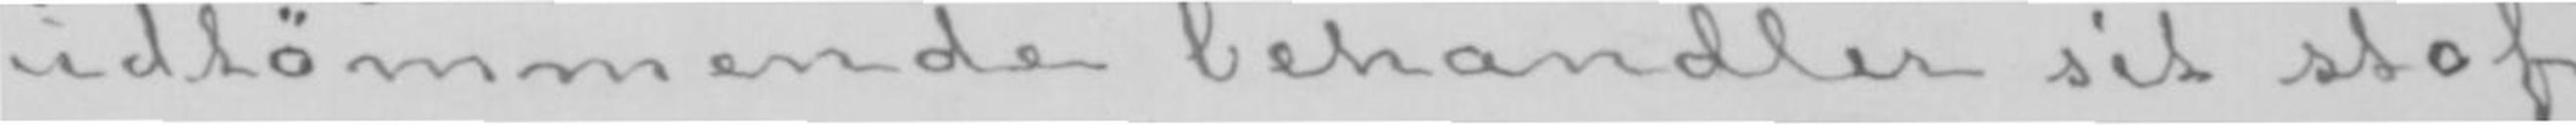udtømmende behandler sit stof
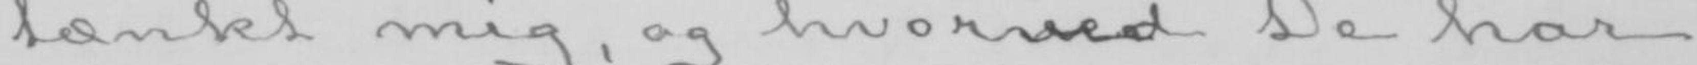tænkt mig, og hvorved De har
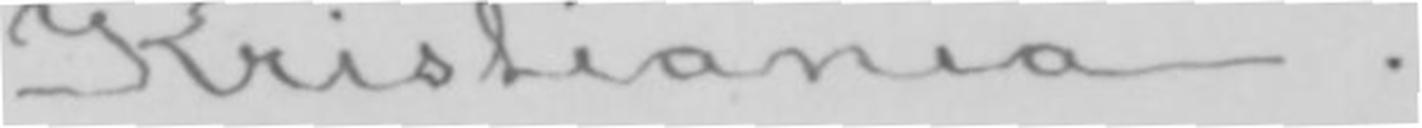Kristiania.
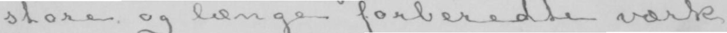store og længe forberedte værk
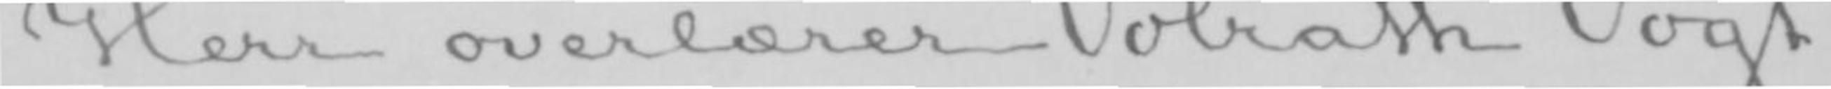Herr overlærer Volrath Vogt.
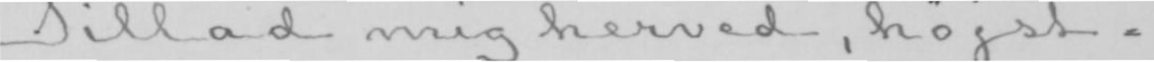Tillad mig herved, højst-
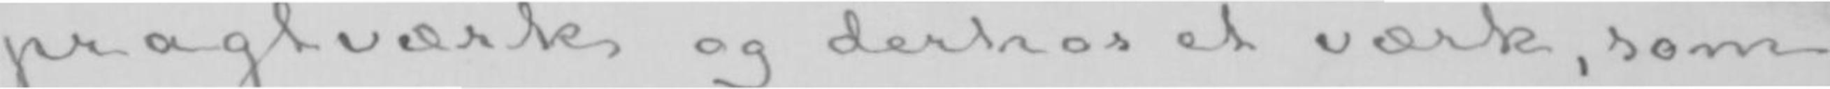pragtværk og derhos et værk, som
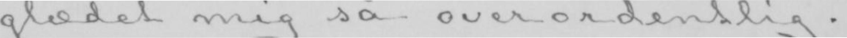glædet mig så overordentlig.
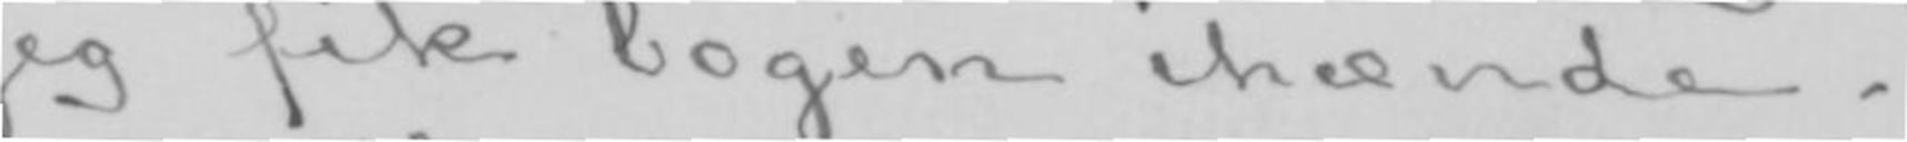jeg fik bogen ihænde.
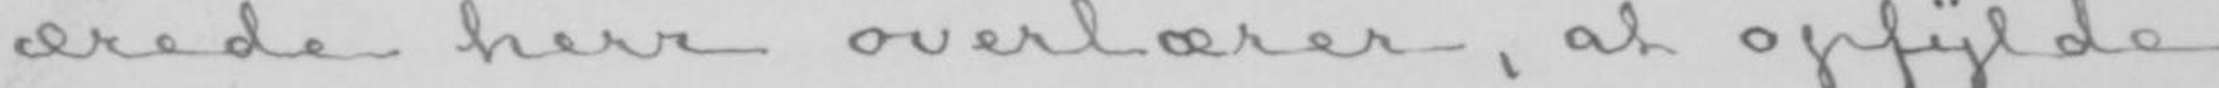ærede herr overlærer, at opfylde
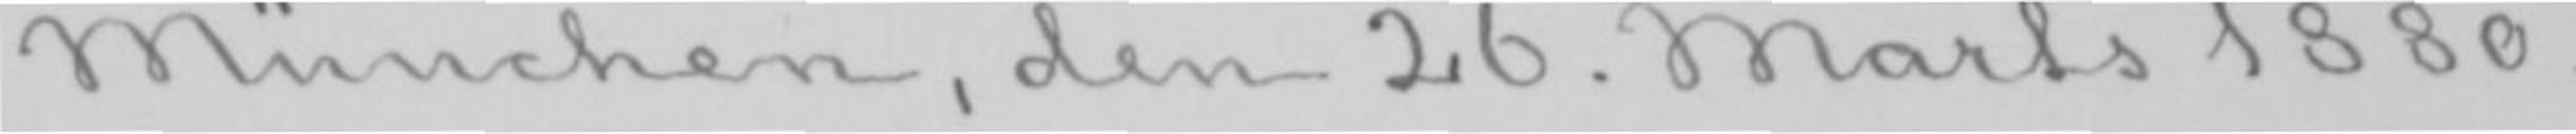München, den 26. Marts 1880.
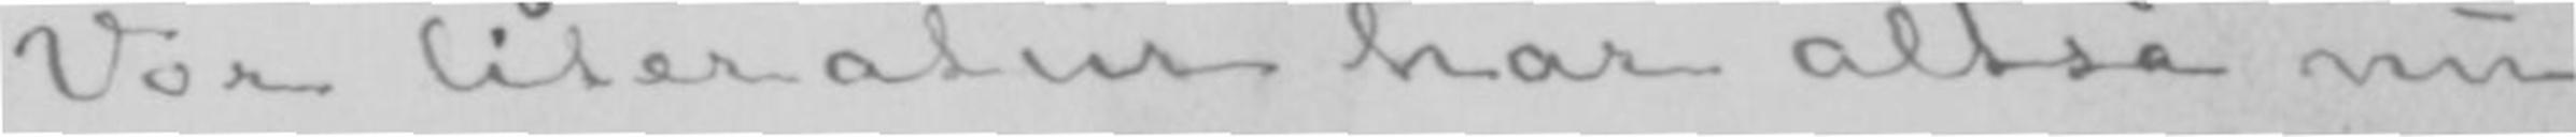Vor literatur har altså nu
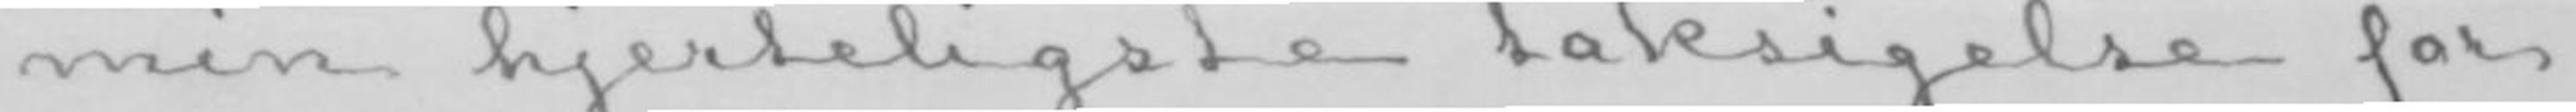min hjerteligste taksigelse for
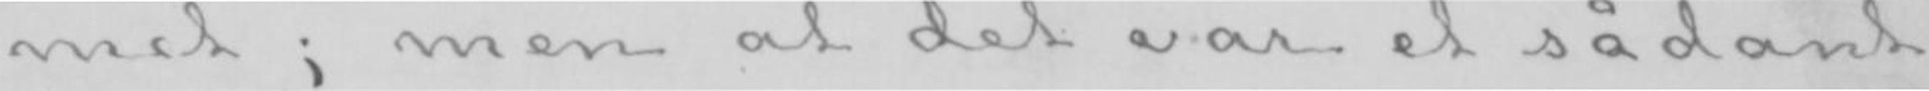met; men at det var et sådant
